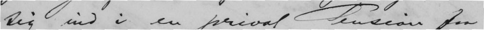sig ind i privat Pension fra
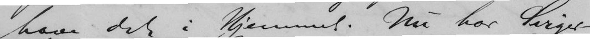have det i Hjemmet. Nu har Sviger-
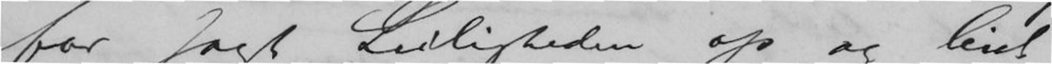far sagt Leiligheden op og leiet
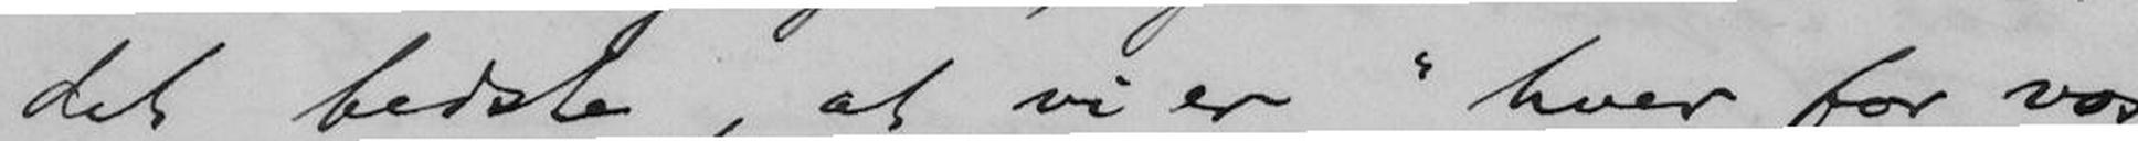det bedste, at vi er "hver for vos".
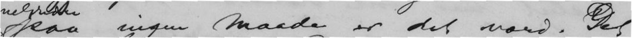paa ingen Maade er det værd. Det
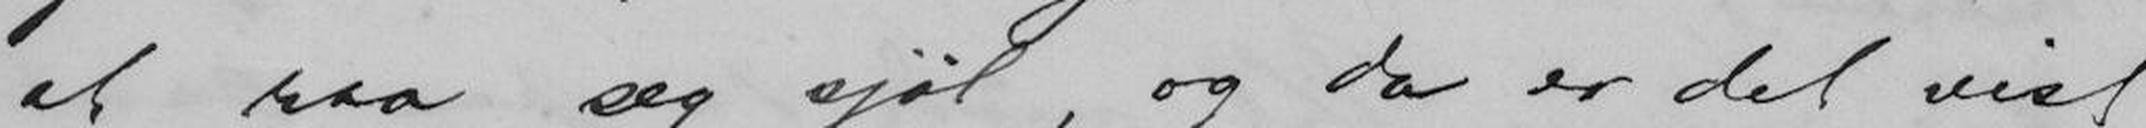at raa seg sjøl, og da er det vist
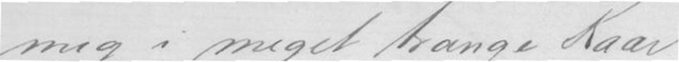mig i meget trange Kaar
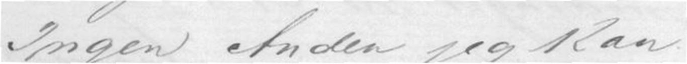Ingen Anden jeg kan
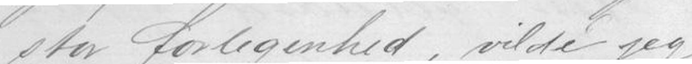stor forlegenhed, vilde jeg
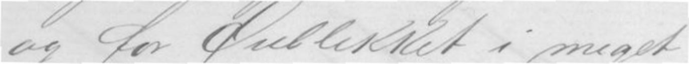og for Øieblikket i meget
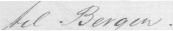til Bergen.
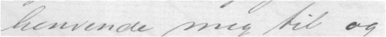henvende mig til og
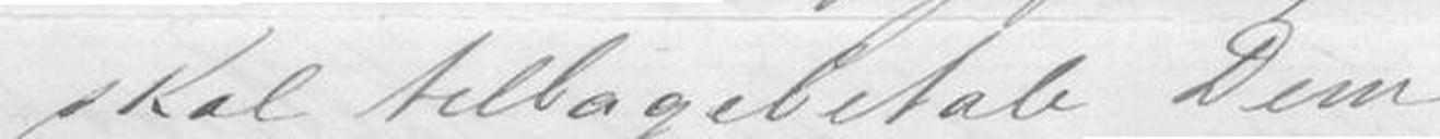skal tilbagebetale Dem
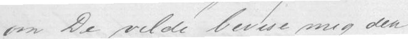om De vilde bevise mig den
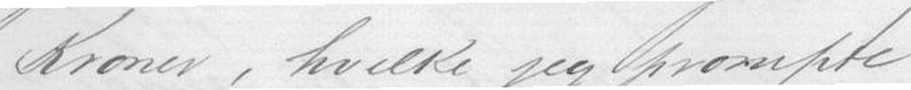Kroner, hvilke jeg prompte
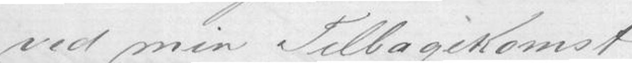ved min Tilbagekomst
